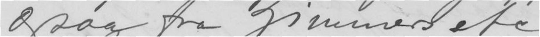ogsaa fra Zimmers etc.
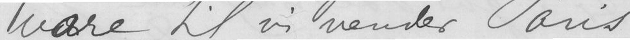være til vi vender Paris
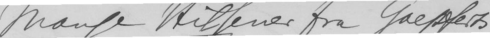Mange Hilsener fra Gaepferts,
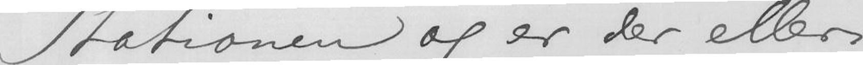Stationen og er der ellers
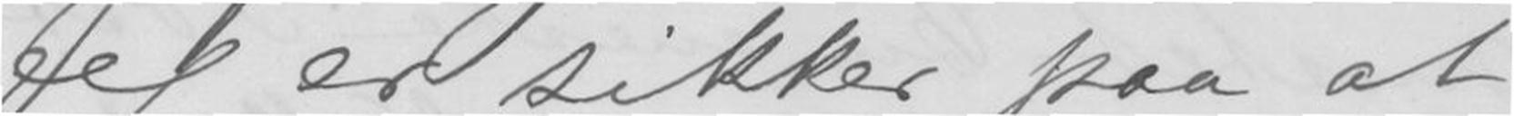Jeg er sikker paa at
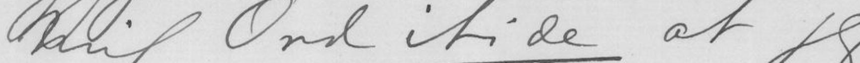mig Ord itide at jeg
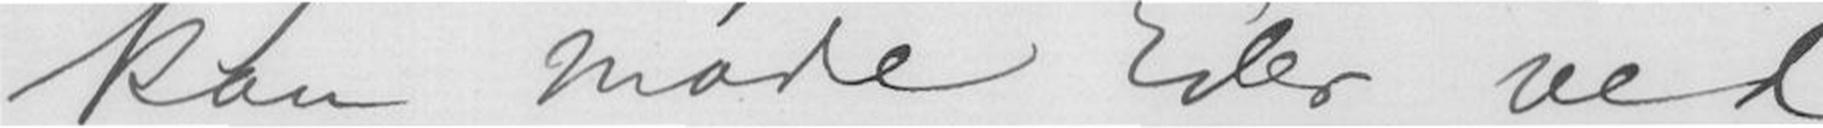kan møde Eder ved
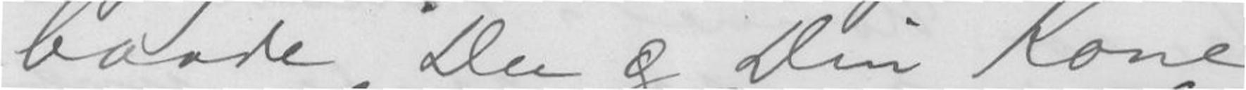baade Du og Din Kone
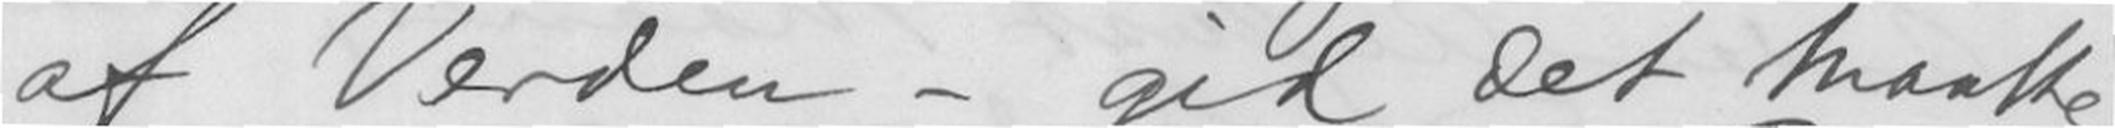af Verden - gid det maatte
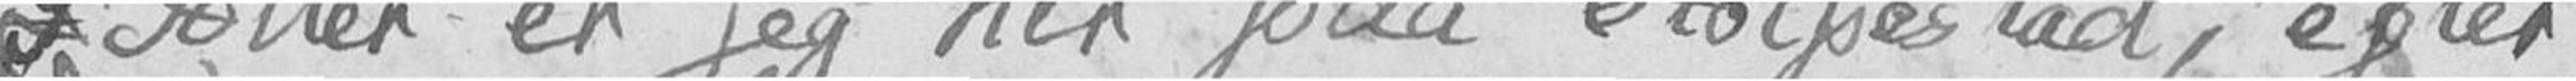I Atter er jeg her paa Stolpestad, efter
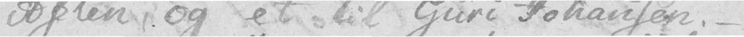Aften, og et til Guri Johansen. –
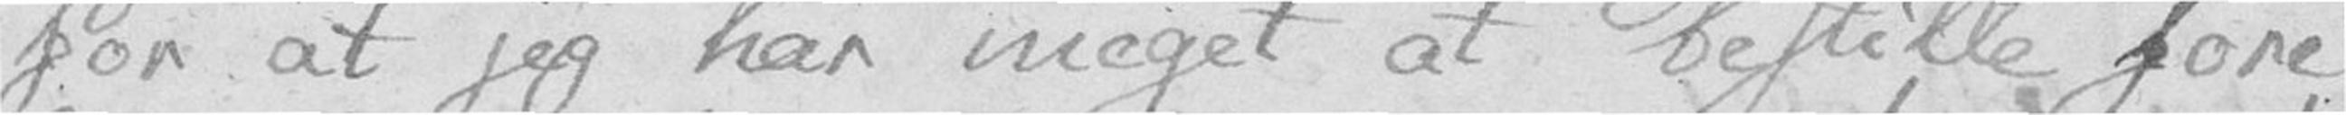for at jeg har meget at bestille fore
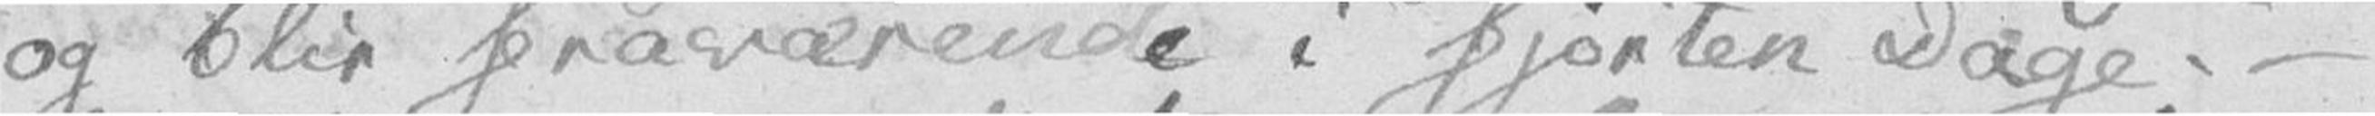og blir fraværende i fjorten Dage. –
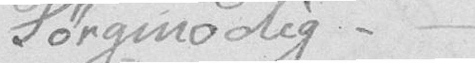Sørgmodig. –
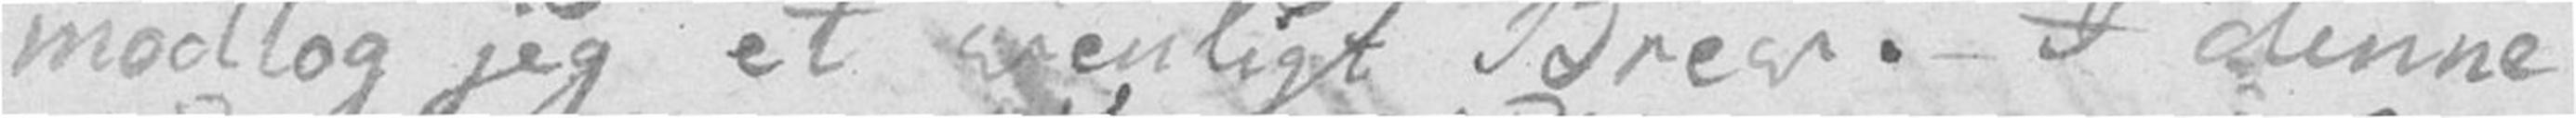modtog jeg et venligt Brev. I denne
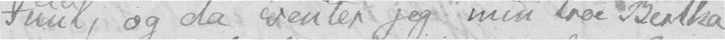Juul, og da venter jeg min troe Bertha
